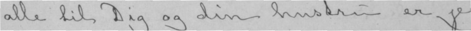alle til Dig og din hustru er jeg
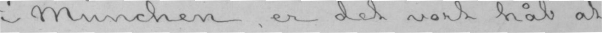i München, er det vort håb at
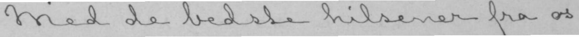Med de bedste hilsener fra os
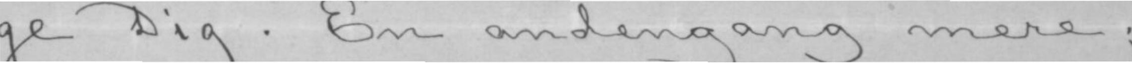ge Dig. En andengang mere;
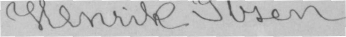Henrik Ibsen.
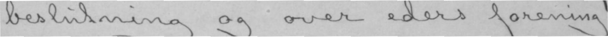beslutning og over eders forening!
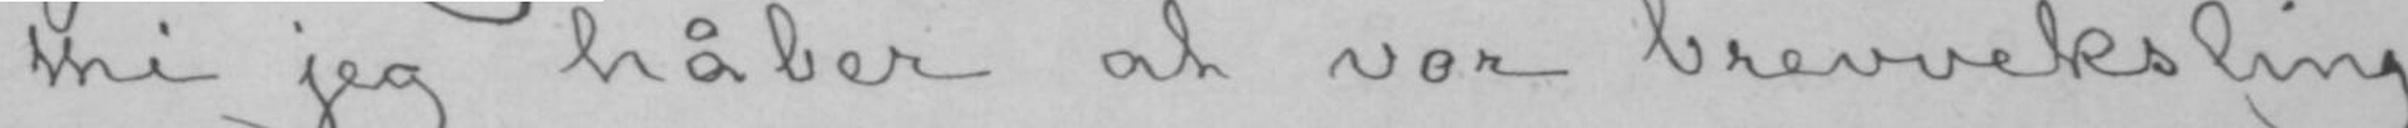thi jeg håber at vor brevveksling
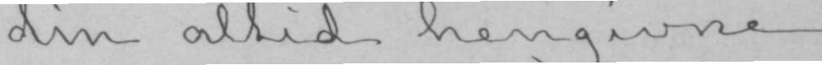din altid hengivne
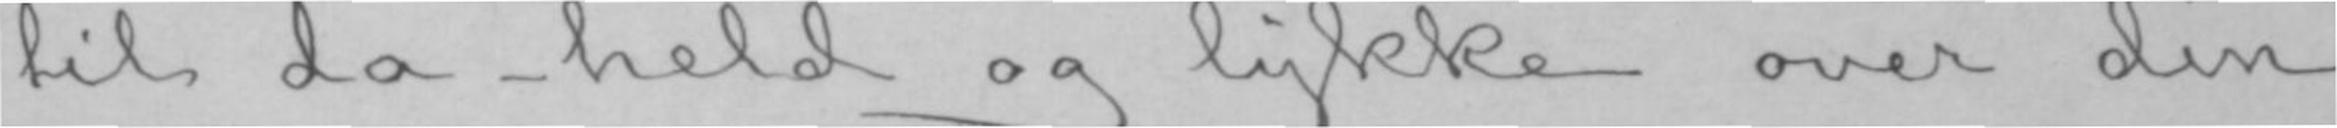til da- held og lykke over din
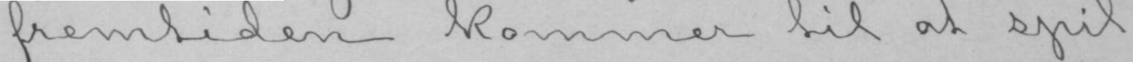fremtiden kommer til at spil-
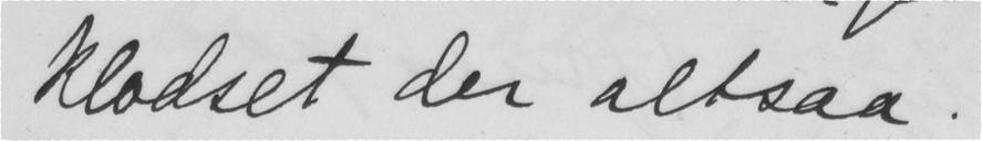klodset der altsaa.
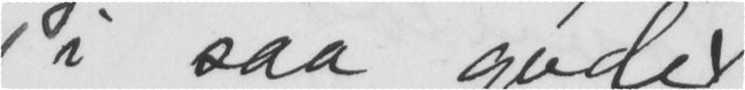i saa gode
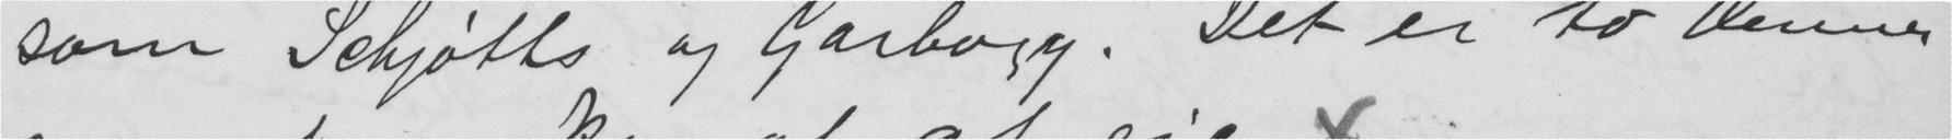som Schjøtts og Garborg. Det er to Venner
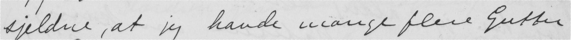sjeldne, at jeg havde mange flere Gutter
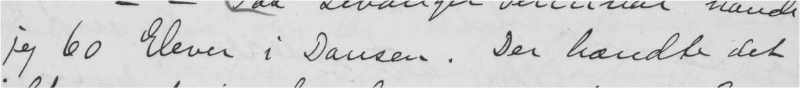jeg 60 Elever i Dansen. Der hændte det
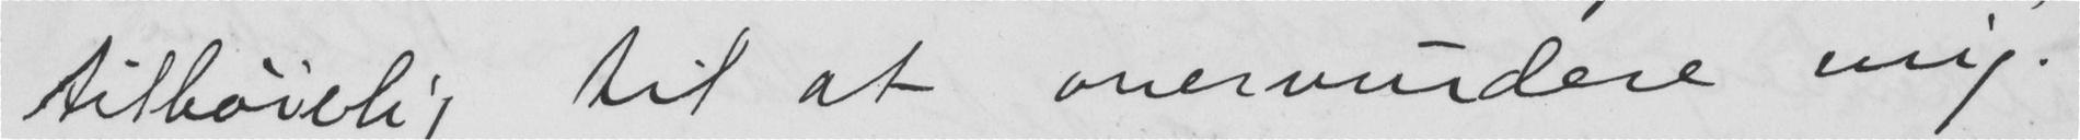tilbøielig til at overvurdere mig.
